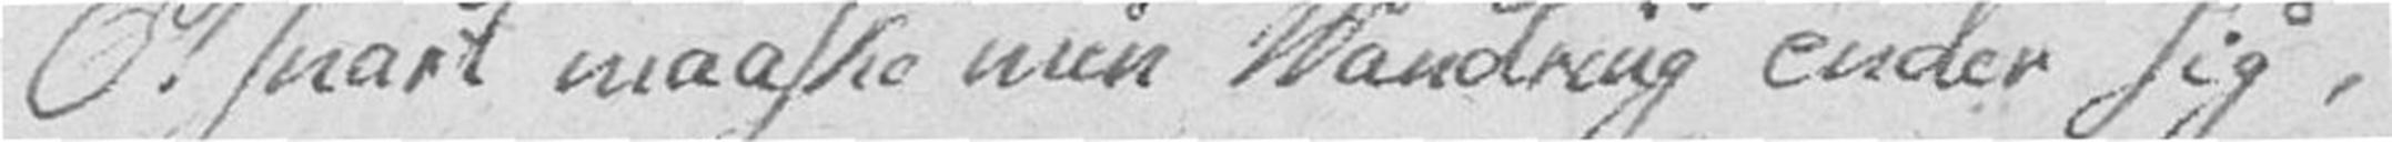O! snart maaske min Wandring ender sig,
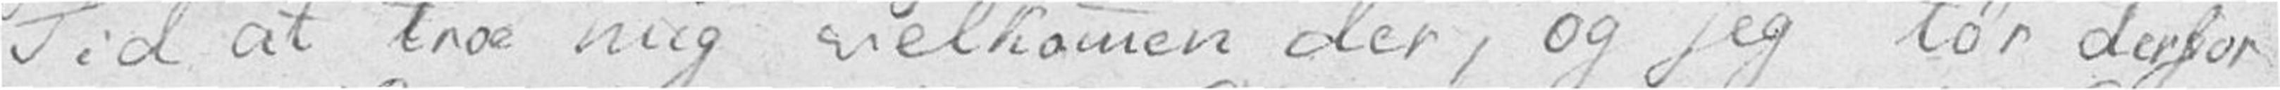Tid at troe mig velkommen der, og jeg tør derfor
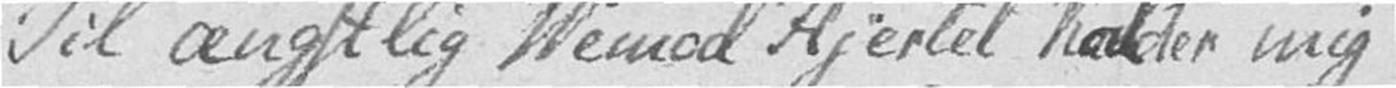Til ængstlig Vemod Hjertet kalder mig
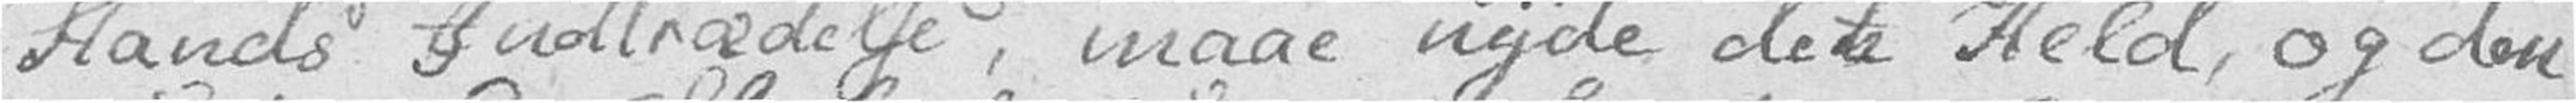Stands Indtrædelse, maae nyde det Held, og den
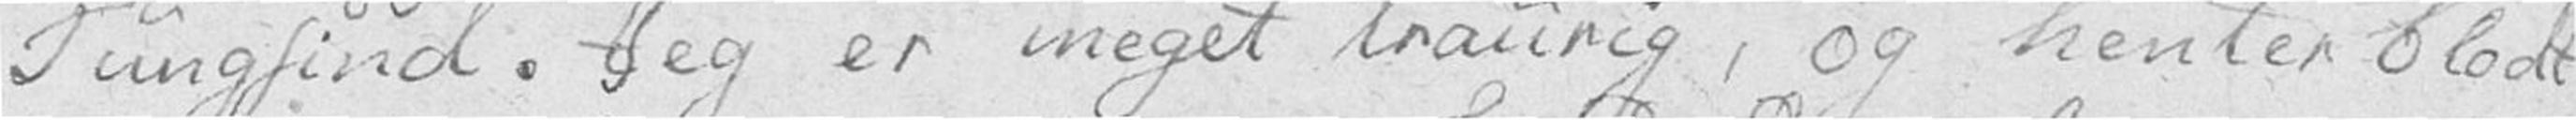Tungsind. Jeg er meget traurig, og henter blodt
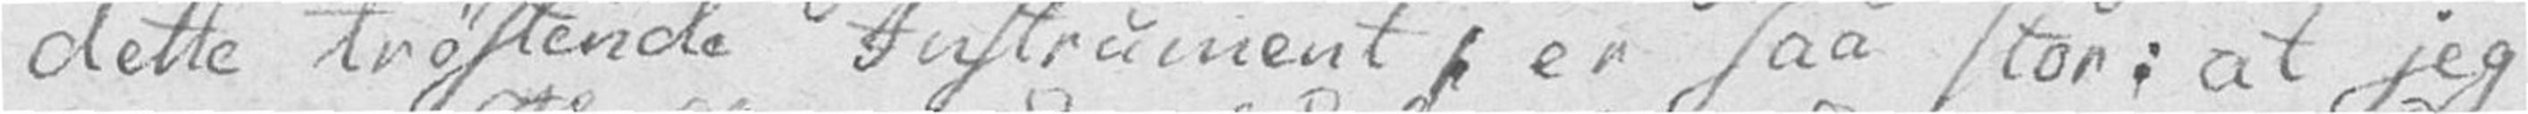dette trøstende Instrument, er saa stor: at jeg
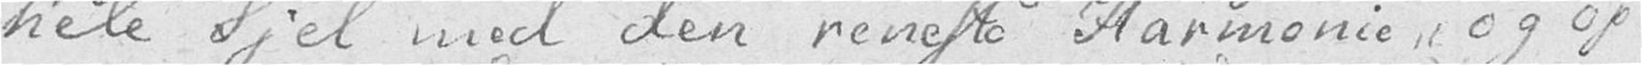hele Sjel med den reneste Harmonie, og op
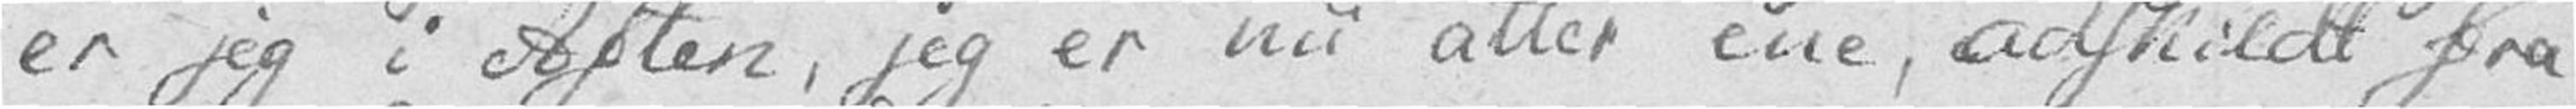er jeg i Aften, jeg er nu atter ene, adskildt fra
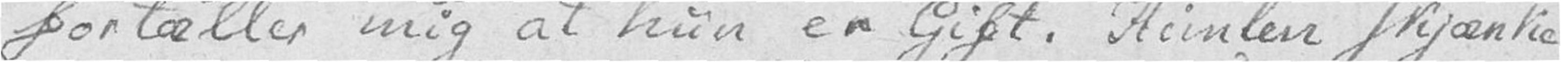fortæller mig at hun er Gift. Himlen skjænke
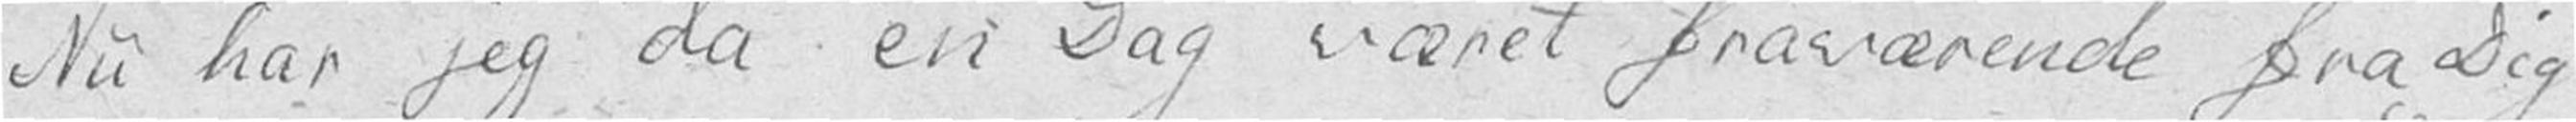Nu har jeg da en Dag været fraværende fra Dig
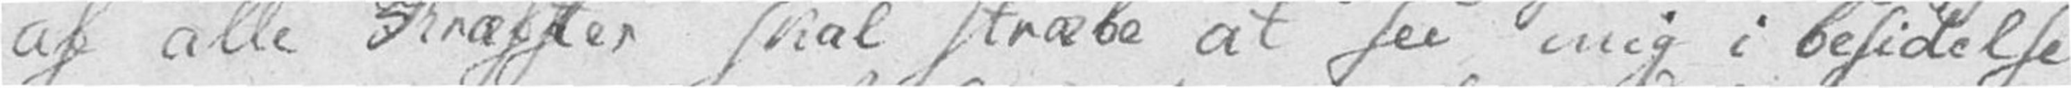af alle Kræfter skal stræbe at see mig i besidelse
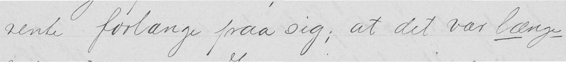vente forlænge paa sig; at det var længe
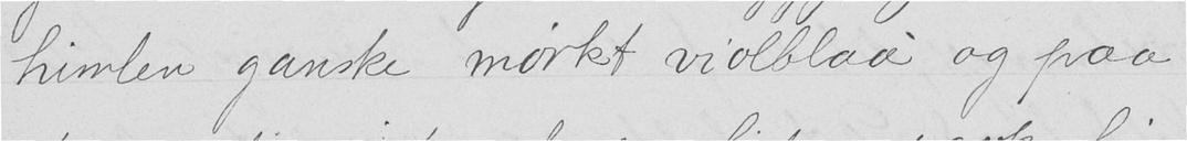himlen ganske mørkt violblaa og paa
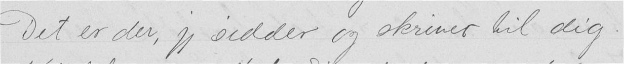Det er der, jeg sidder og skriver til dig.
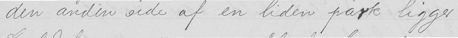den anden side af en liden park ligger
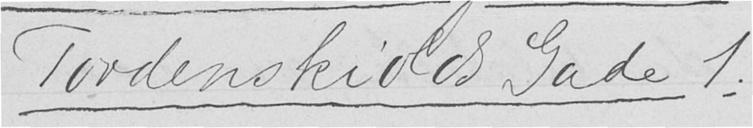Tordenskiolds Gade 1!
